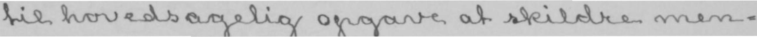til hovedsagelig opgave at skildre men-
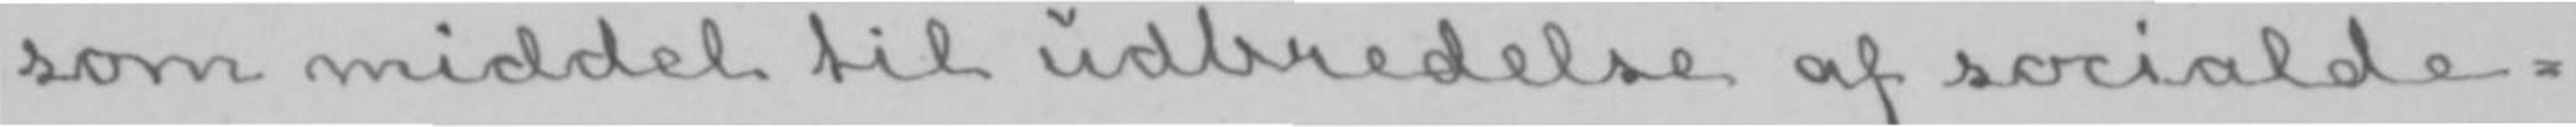som middel til udbredelse af socialde-
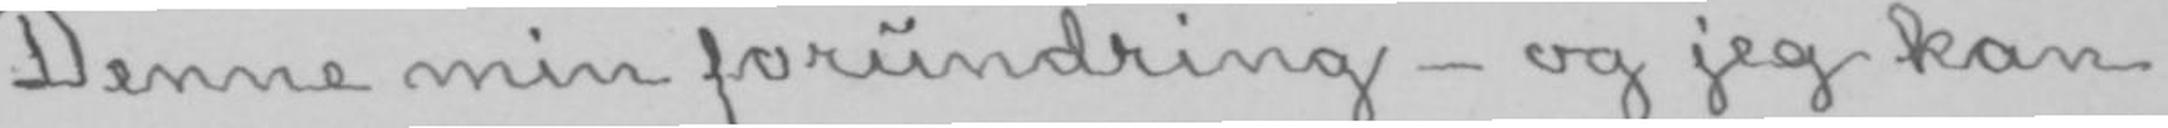Denne min forundring- og jeg kan
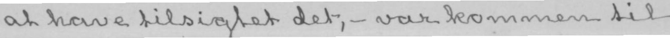at have tilsigtet det;- var kommen til
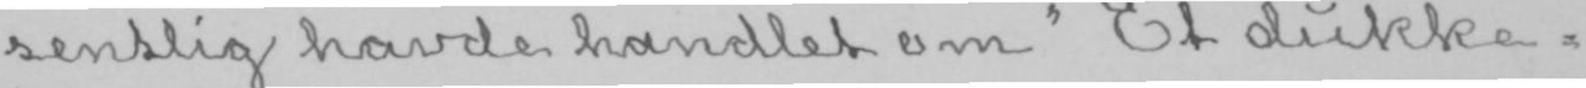sentlig havde handlet om «Et dukke-
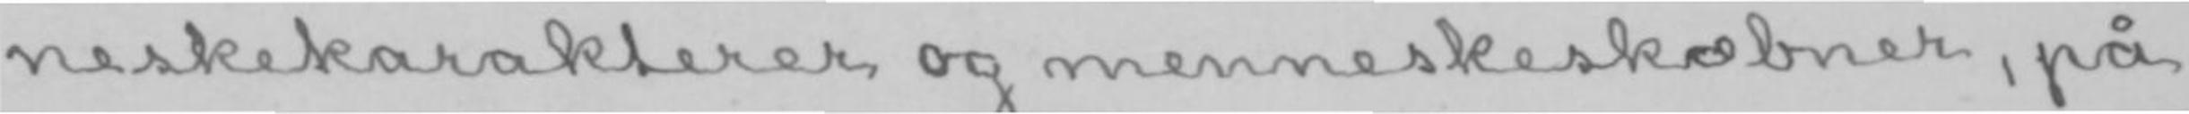neskekarakterer og menneskeskæbner, på
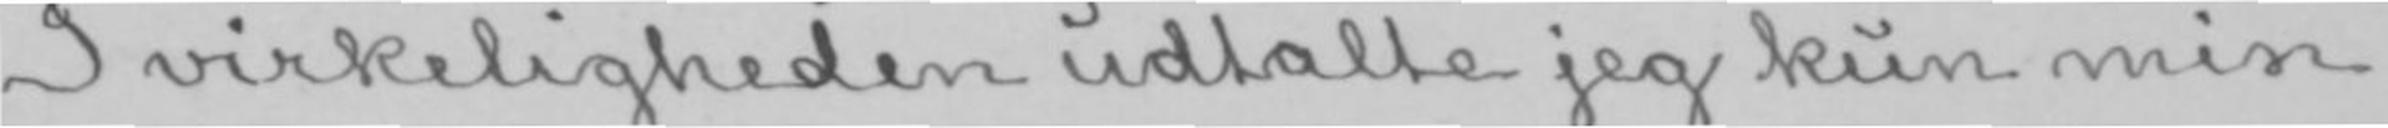I virkeligheden udtalte jeg kun min
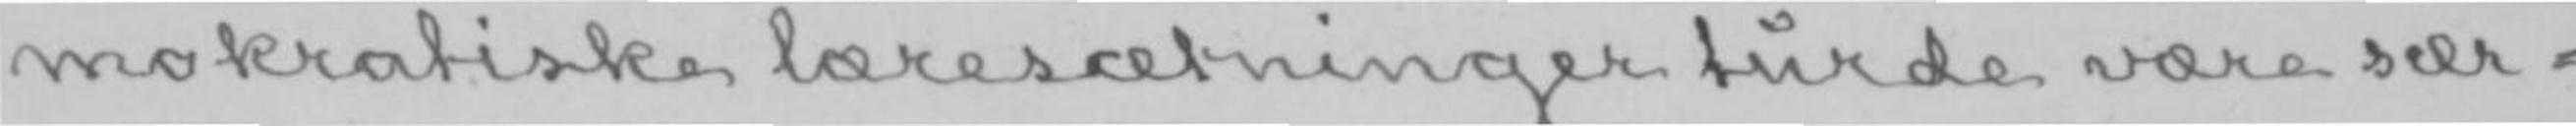mokratiske læresætninger turde være sær-
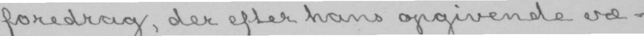foredrag, der efter hans opgivende væ-
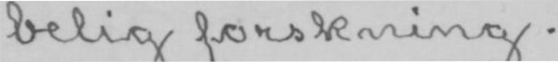belig forskning.
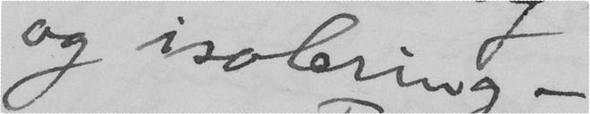og isolering –
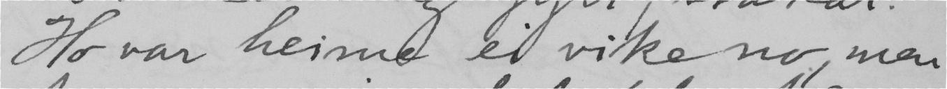Ho var heime ei vike no, men
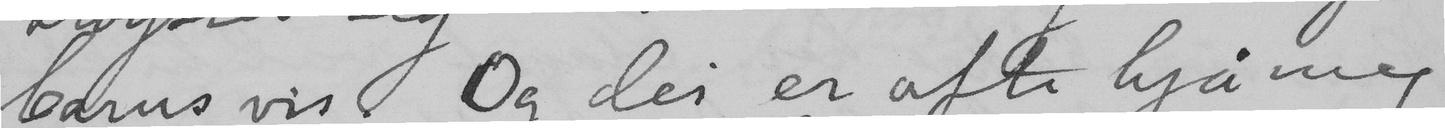barns vis. Og dei er ofte hjå meg
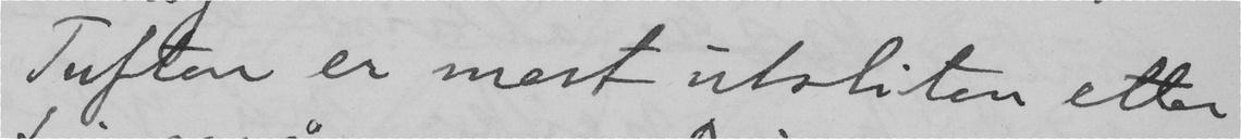Tuften er mest utsliten etter
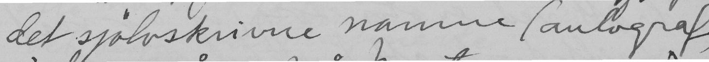det sjölvskrivne namne (autograf)
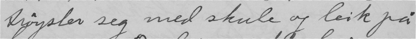tröyster seg med skule og leik på
